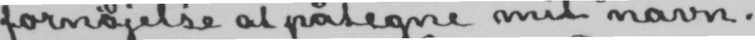fornøjelse at påtegne mit navn.
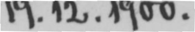19.12.1900.
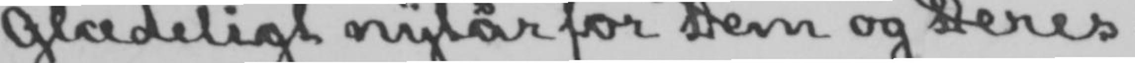Glædeligt nytår for Dem og Deres.
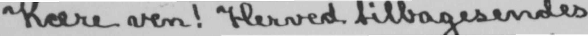Kære ven! Herved tilbagesendes
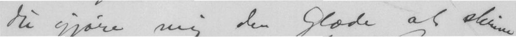du gjøre mig den Glæde at skrive
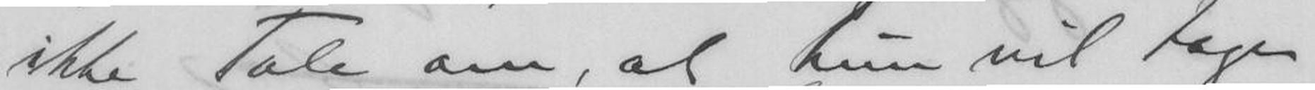ikke Tale om, at hun vil tage
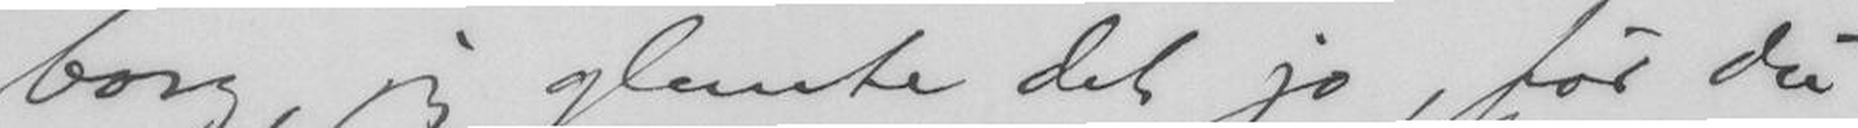borg, jeg glemte det jo, før du
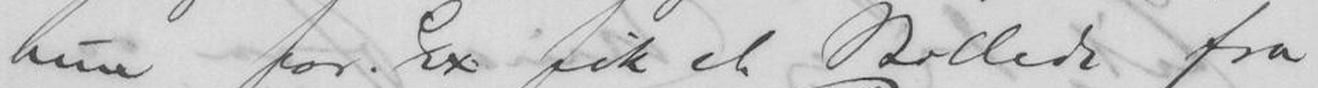hun for Ex fik et Billede fra
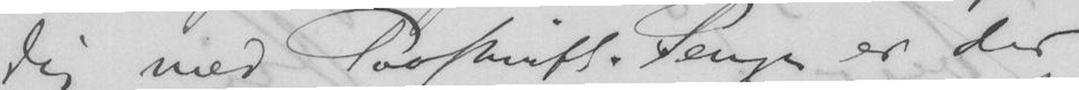dig med Paaskrift. Penge er der
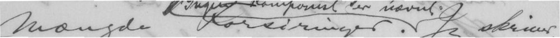Mængde Forsiringer. Jeg skriver
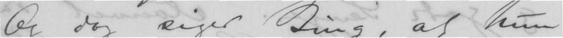Og dog siger Bing, at hun
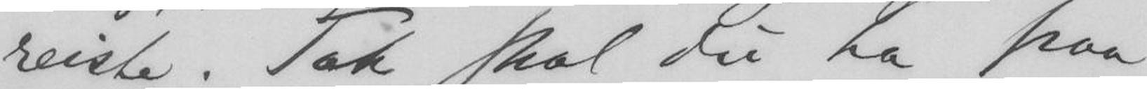reiste. Tak skal du ha paa
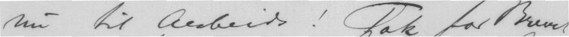nu til Arbeids! Tak for Brevet
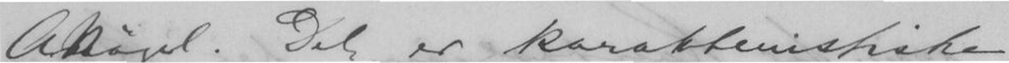ANøgel. Det er karakteristiske,
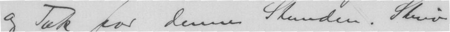og Tak for denne Stunden. Skriv
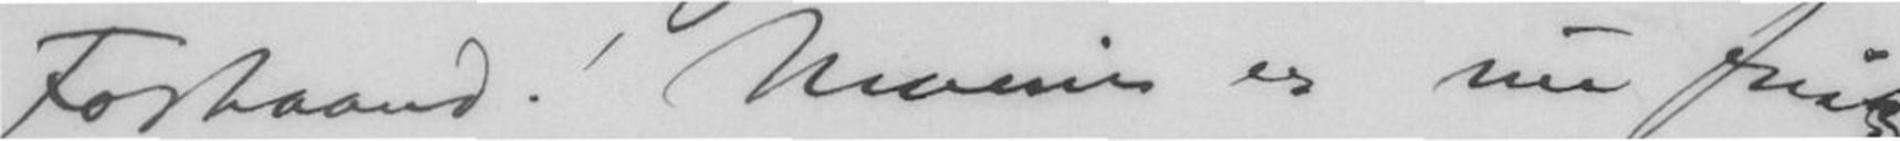Forhaand! Maven ? Marie?? er nu frisk
